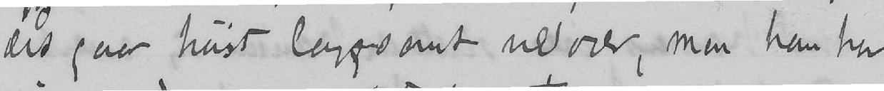det gaar høist langsomt nedover, men han har
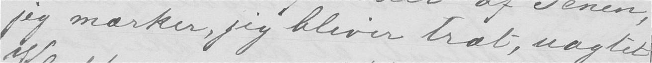jeg mærker, jeg bliver træt, uagtet
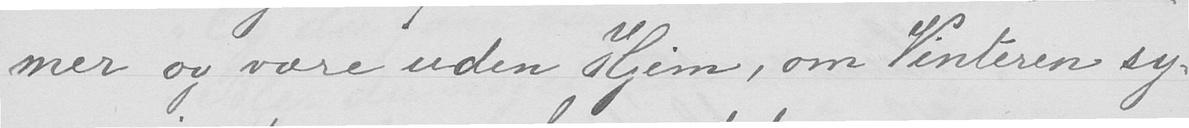mer og være uden Hjem, om Vinteren sy-
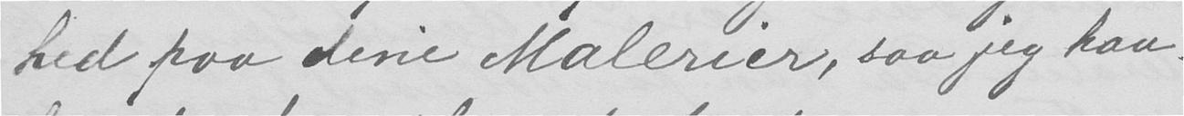hed paa dine Malerier, saa jeg haa-
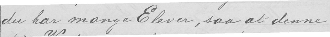du har mange Elever, saa at denne
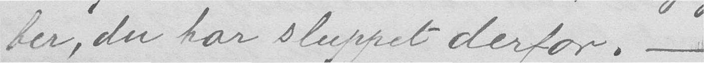ber, du har sluppet derfor. -
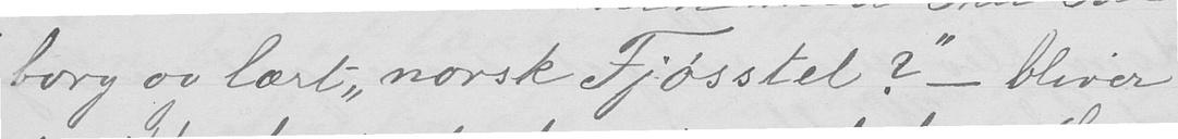borg og lært "norsk Fjøsstel?" - bliver
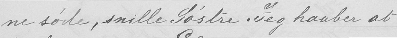ne søde, snille Søstre. Jeg haaber at
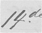14de
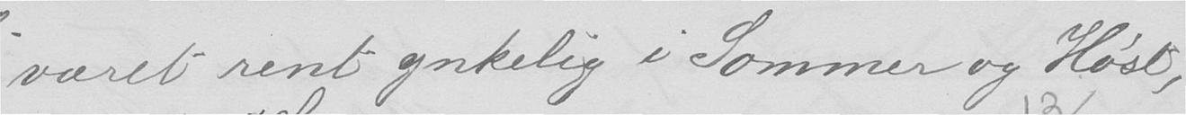været rent ynkelig i Sommer og Høst,
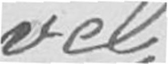vel
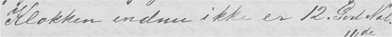Klokken endnu ikke er 12. God Nat.
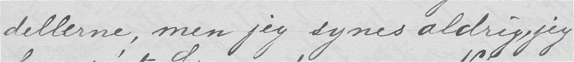dellerne, men jeg synes aldrig, jeg
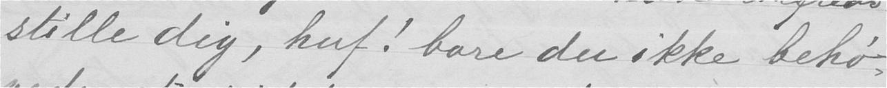stille dig, huf! bare du ikke behø-
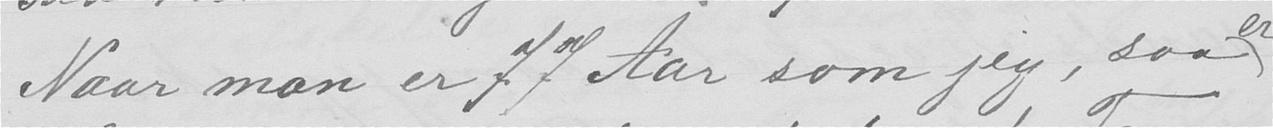Naar man er 77 Aar som jeg, saa er
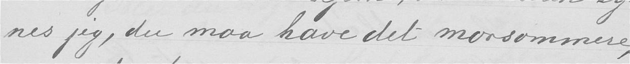nes jeg, du maa have det morsommere,
